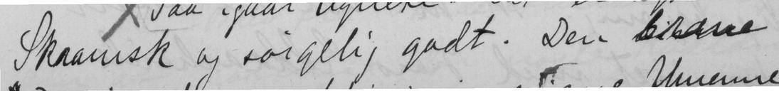Skramsk og sørgelig godt. Den bisarre
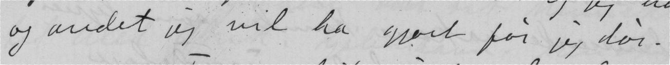og andet jeg vil ha gjort før jeg dør.
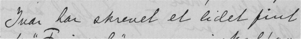Ivar har skrevet et lidet fint
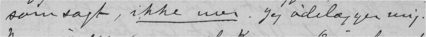som sagt, ikke mer. Jeg ødelægger mig.
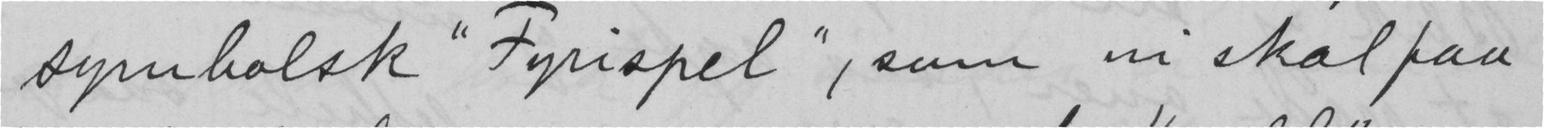symbolsk "Fyrispel", som vi skal faa
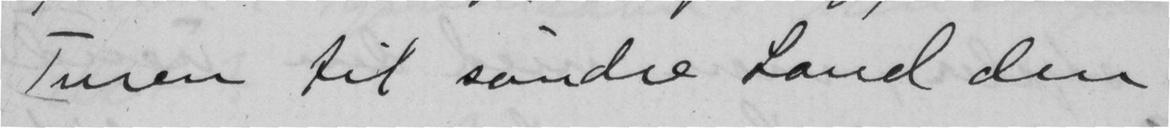Turen til søndre Land den
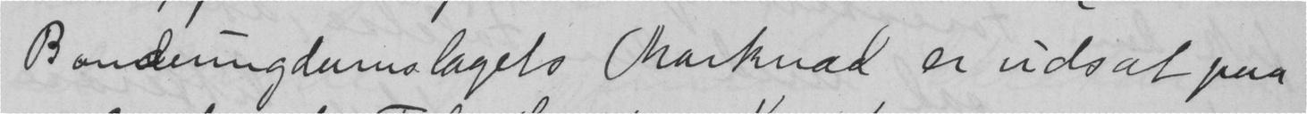Bondeungdomslagets Marknad er udsat paa
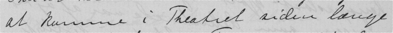at komme i Theatret siden længe
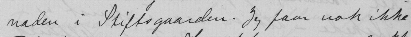naden i Stiftsgaarden. Jeg faar nok ikke
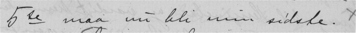5te maa nu bli min sidste. x
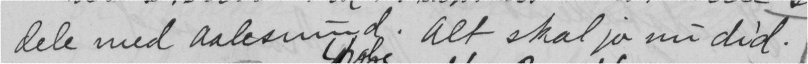dele med Aalesund Alt skal jo nu did.
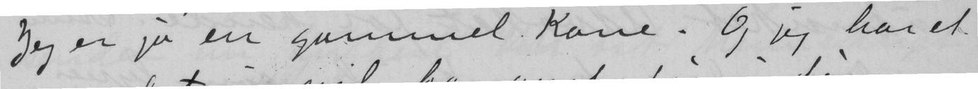Jeg er jo en gammel Kone. Og jeg har et
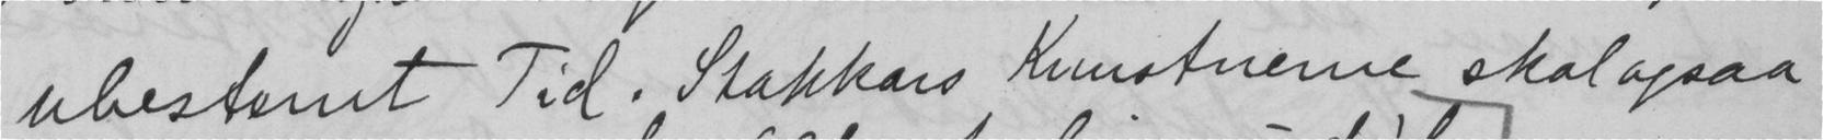ubestemt Tid. Stakkars Kunstnerne skal ogsaa
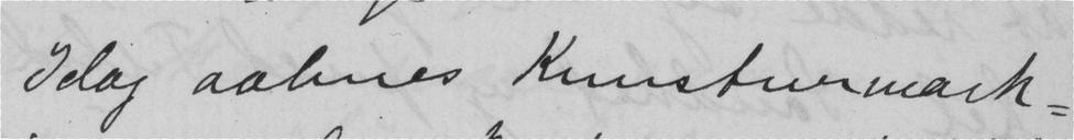Idag aabnes Kunstnermark-
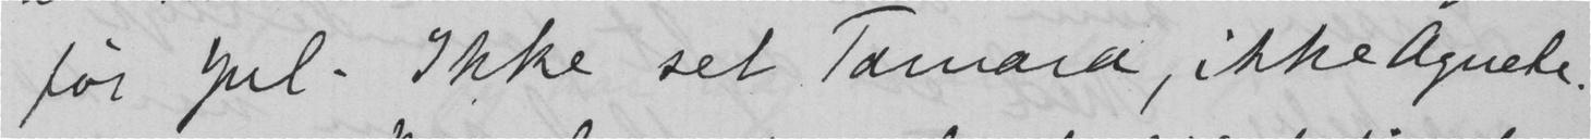før Jul. Ikke set Tamara, ikke Agnete.
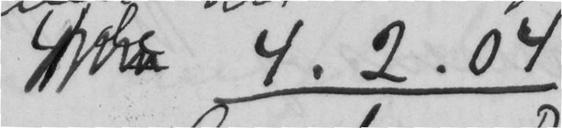4.2.04
